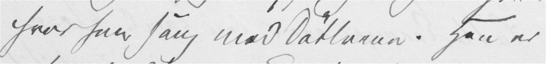hvor han sang med Døttrene. Han er
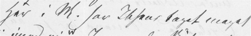Her i M. har Ibsens taget meget
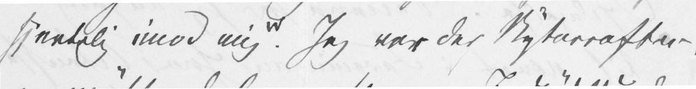hjertelig imod mig. Jeg var der Nytarraften,
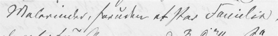Malerinder, foruden et Par Familier,
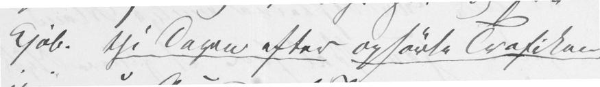Kjøb. thi Dagen efter ophørte Trafiken
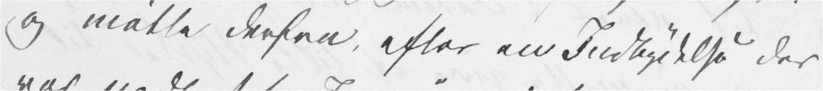og måtte derfra, efter en Indbydelse der
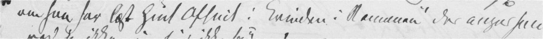om han har læst Hint Afsnit i Kvinden i Romanen" der angår ham
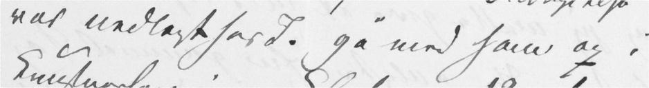var nedlagt hos I. gå med ham op i
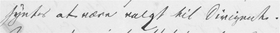syntes at være valgt til Dirigent.
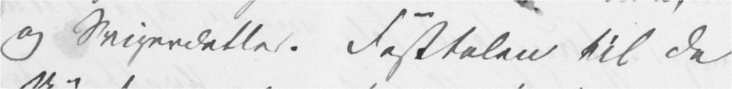og Svigerdatter. Festtalen til de
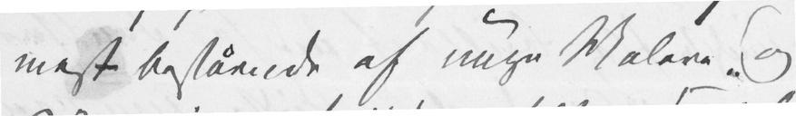mest bestående af unge Malere og
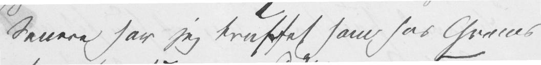Senere har jeg truffet ham hos Grams
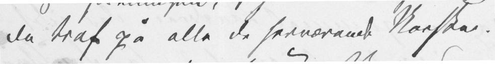da traf på alle de herværende Norske.
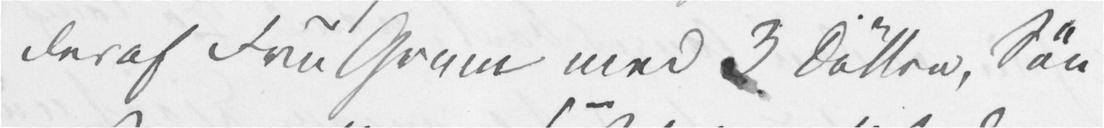deraf Fru Gram med 3 Døttre, Søn
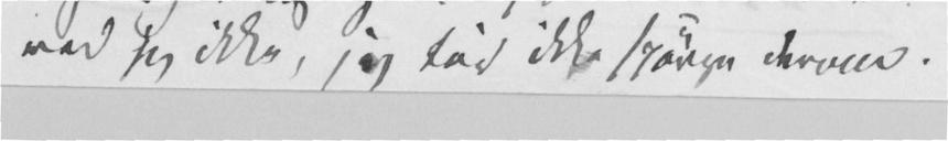ved jeg ikke, jeg tør ikke spørge derom.
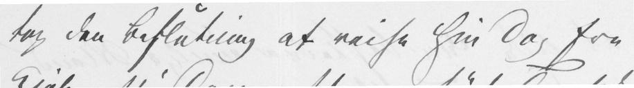tog den Beslutning at reise Hin Dag fra
